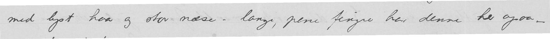med lyst haar og stor næse – lange, pene fingre har denne her ogsaa –
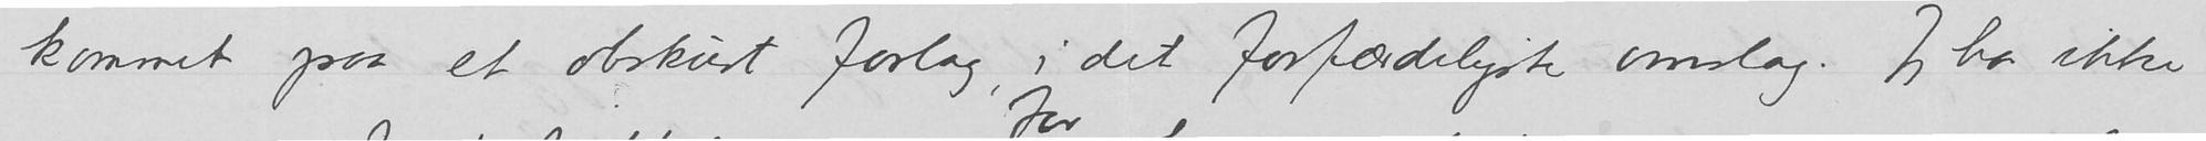kommet paa et obskurt forlag, i det forfærdeligste omslag. Jeg har ikke
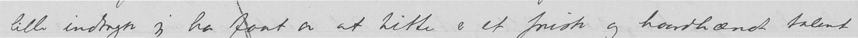lille indtryk jeg har faat av at titte er et frisk og haardhændt talent
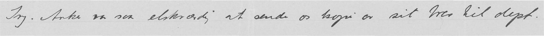Ing. Anker var saa elskværdig at sende os kopi av sit brev til dept.
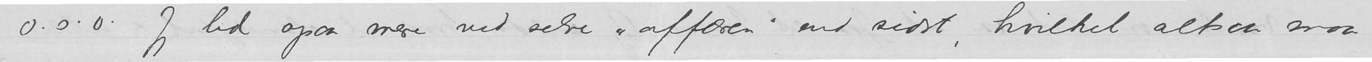o.s.v. Jeg led ogsaa mere ved selve i «affæren» end sidst, hvilket altsaa maa
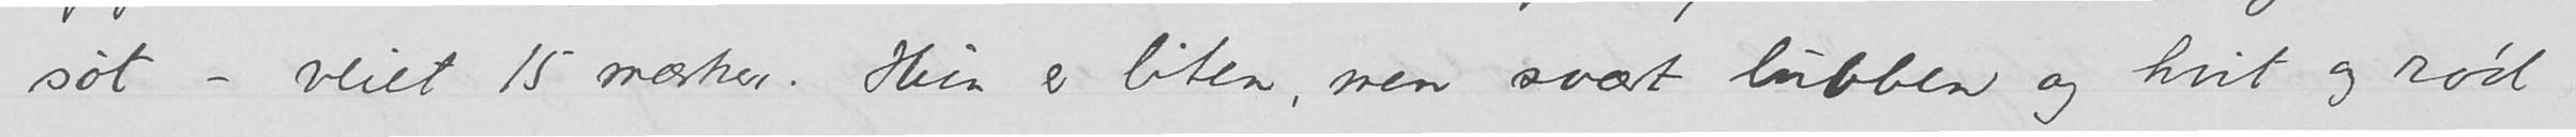søt – veiet 15 mærker. Hun er liten, men svært lubben og hvit og rød
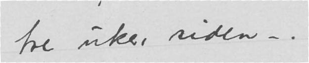tre uker siden –.
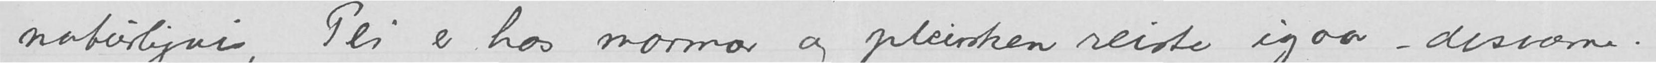naturligvis, Pei er hos mormor og pleiersken reiste igaar – desværre.
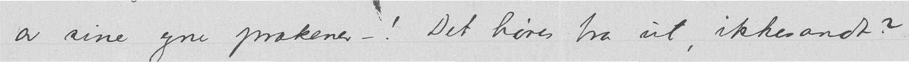av sine egne prækener –! Det høres bra ut, ikkesandt?
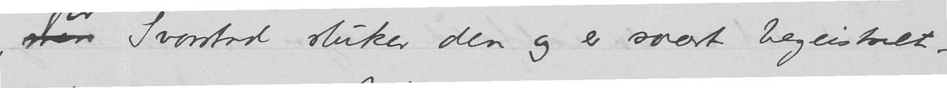men Svarstad sluker den og er svært begeistret –
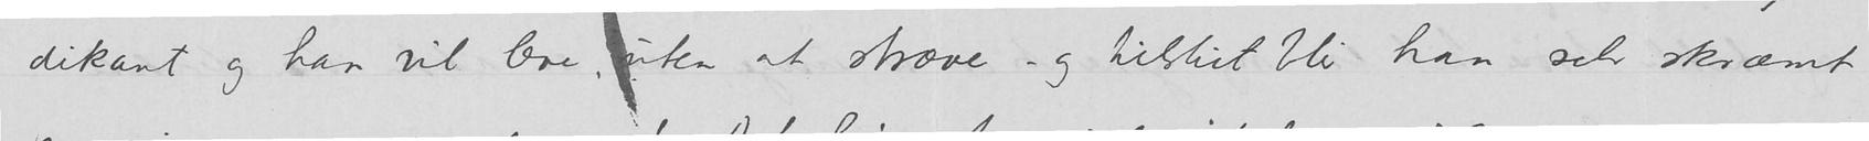dikant og han vil leve uten at stræve – og tilslut blir han selv skræmt
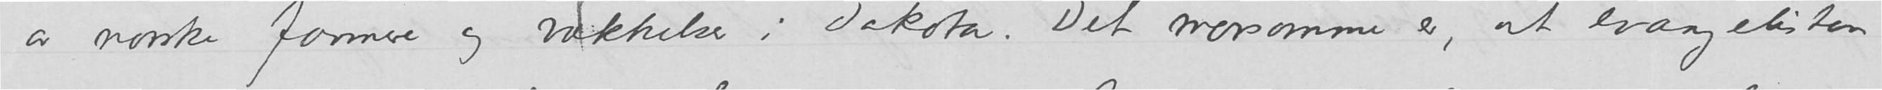av norske farmere og vækkelser i Dakota. Det morsomme er, at evangelisten
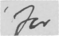for
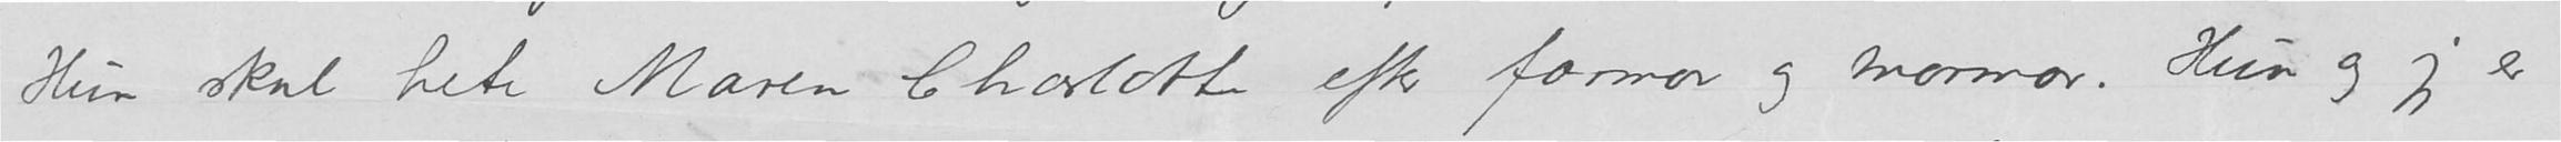Hun skal hete Maren Charlotte efter farmor og mormor. Hun og jeg er
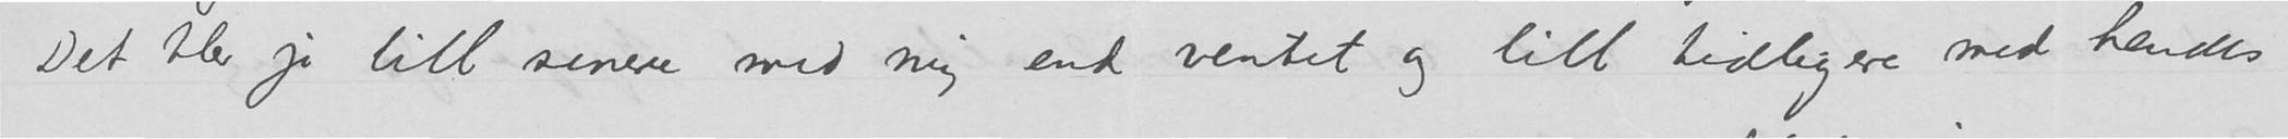Det blev jo litt senere med mig end ventet og litt tidligere med hendes
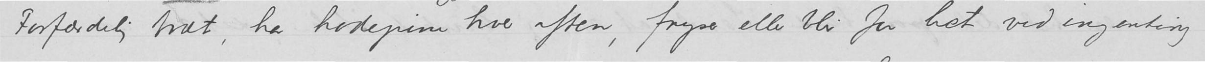Forfærdelig træt, har hodepine hver aften, fryser eller blir for het ved ingenting
